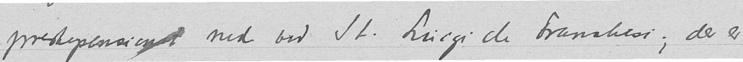prestepensionat ned ved St. Luigi de Franchesi; der er
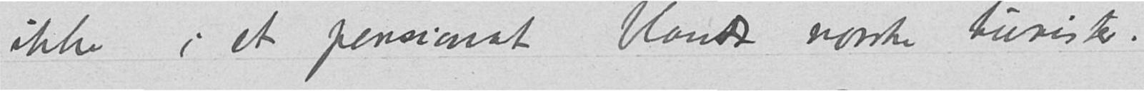ikke i et pensionat blandt norske turister.
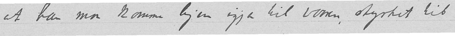at han maa komme hjem igjen til vaaren, styrket til
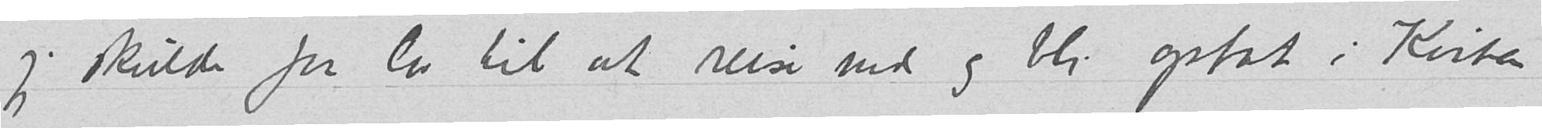jeg skulde faa lov til at reise ned og bli optat i Kirken
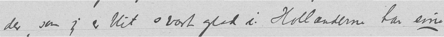der, som jeg er blit svært glad i. Hollænderne har sine
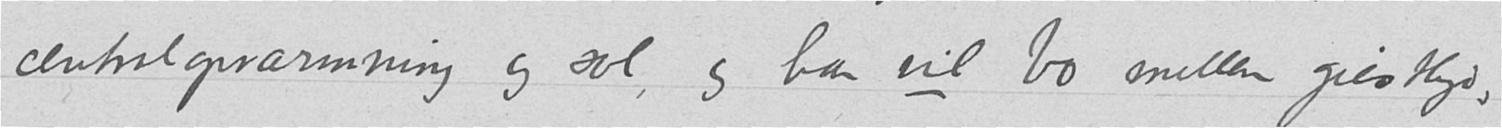centralopvarmning og sol, og han vil bo mellem geistlige,
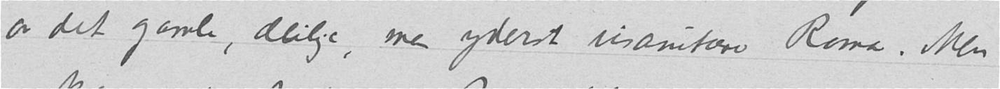av det gamle, deilige, men yderst usanitære Roma. Men
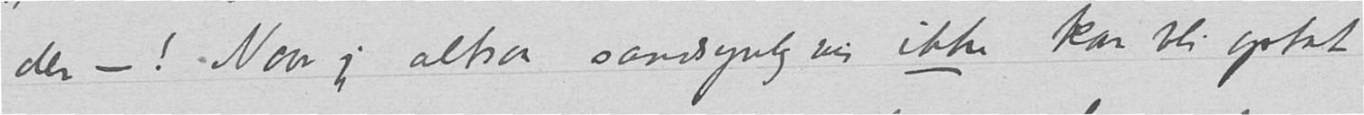der -!  Naar jeg altsaa sandsynligvis ikke kan bli optat
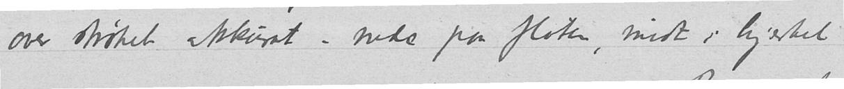over strøket akkurat – nede paa flaten, midt i hjertet
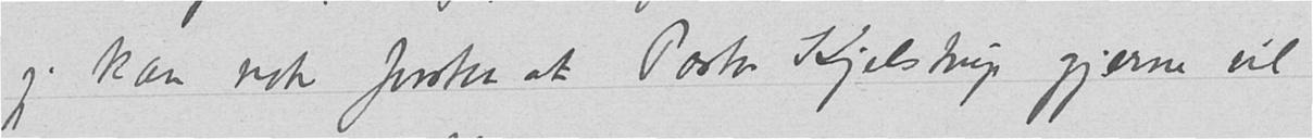jeg kan nok forstaa at Pater Kjelstrup gjerne vil
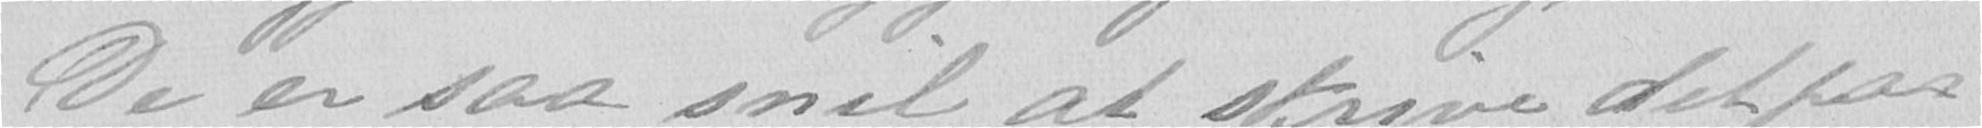De er saa snil at skrive det paa
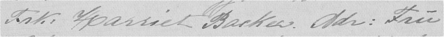Frk. Harriet Backer. Adr. Fru
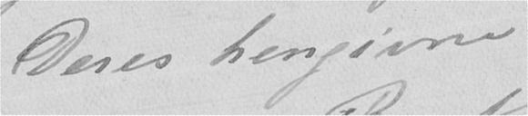Deres hengivne
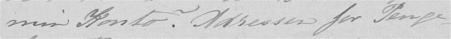min Konto? Adressen for Penge-
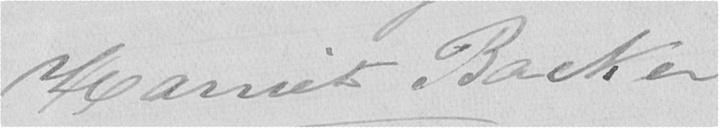Harriet Backer
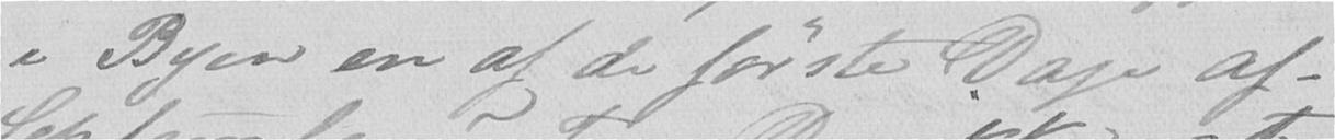i Byen en af de første Dage af
